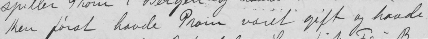Men først havde Prom været gift og havde
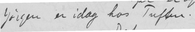Jørgen er idag hos Tuften.
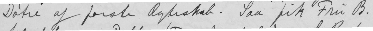Døtre af første Ægteskab. Saa fik Fru B.
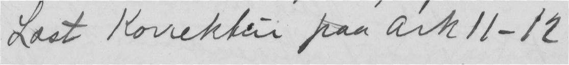Læst Korrektur paa Ark 11-12
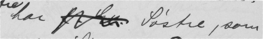har  Søstre, som
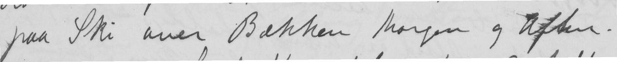paa Ski over Bækken Morgen og Aften.
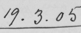19.3.05
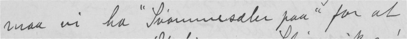maa vi ha "Svømmesæler paa" for at
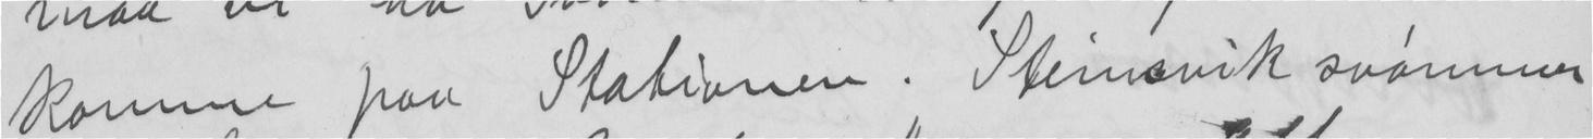komme paa Stationen. Steinsvik svømmer
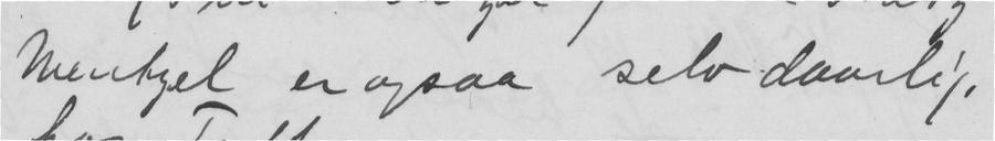Wentzel er ogsaa selv daarlig.
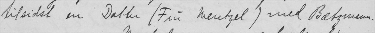tilsidst en Datter (Fru Wentzel) med Bætzmann.
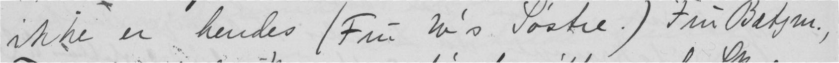ikke er hendes (Fru W's Søstre) Fru Bætzm.,
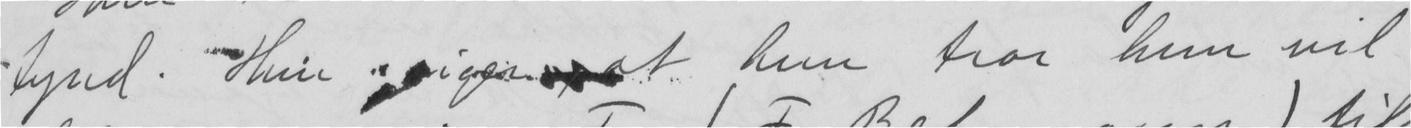Synd. Hun siger at hun tror hun vil
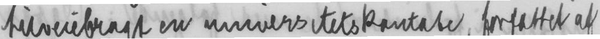tilveiebragt en universitstskantate, forfattet af
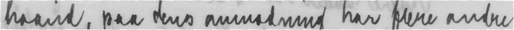haand, paa dens anmodning har flere andre
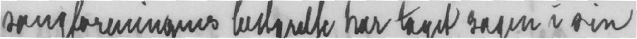sangforeningens bestyrelse har taget sagen i sin
